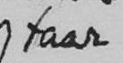faar
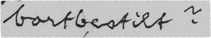bortbestilt?
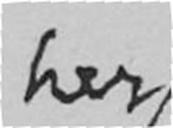her
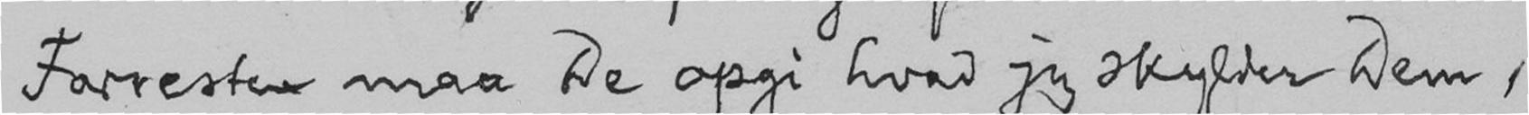Forresten maa De opgi hvad jeg skylder Dem,
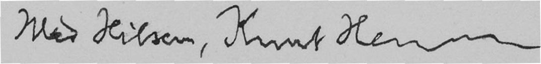Med Hilsen, Knut Hamsun
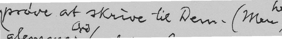prøve at skrive til Dem. (Men
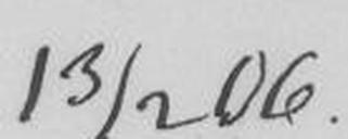13/2 06.
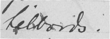tilbords.
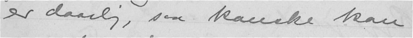er daarlig, saa kanske kan
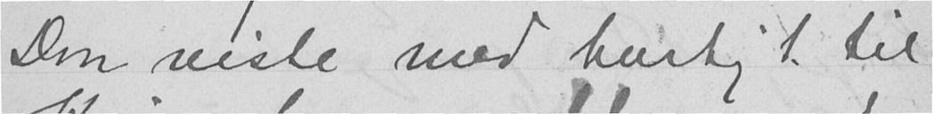Don reiste med hurtig t. til
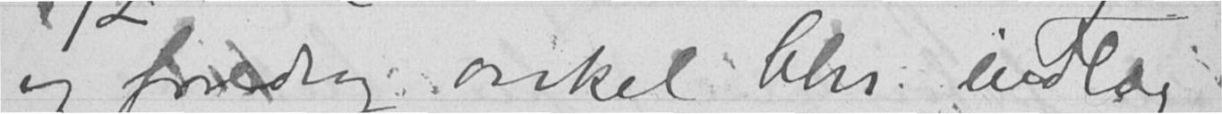og foredrog onkel Chr. indlæg
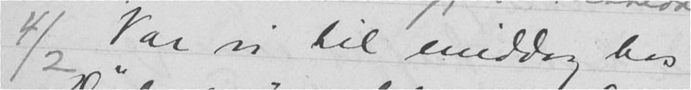4/2 Var vi til middag hos
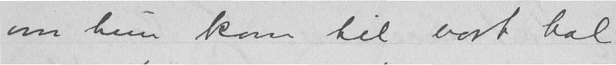om hun kom til vort bal
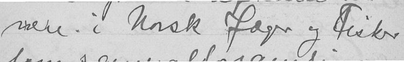være i Norsk Jæger og Fisker
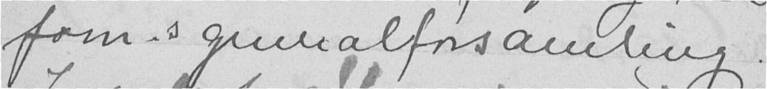forn.s generalforsamling.
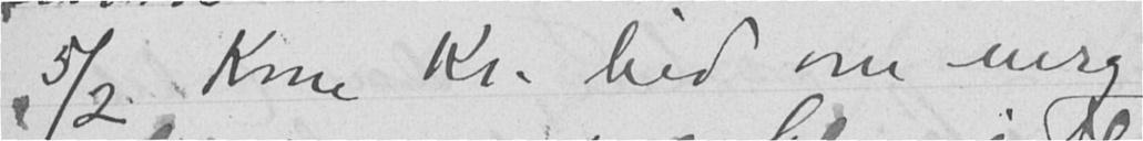5/2 Kom Kr. hid om mrg
